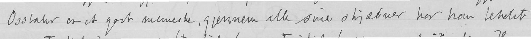Ossbahr er et godt menneske, gjennem alle sine skjæbner har han beholdt
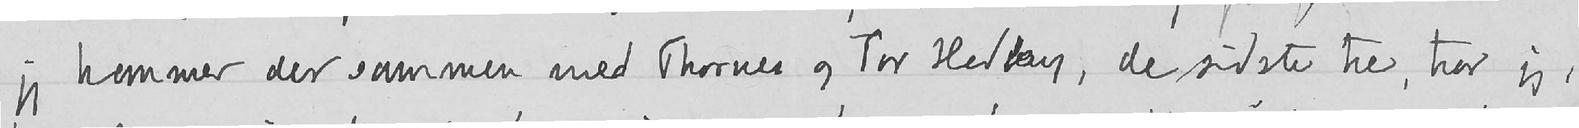jeg kommer der sammen med Thornes og Tor Hedberg, de sidste tre, tror jeg,
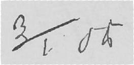3/1 05
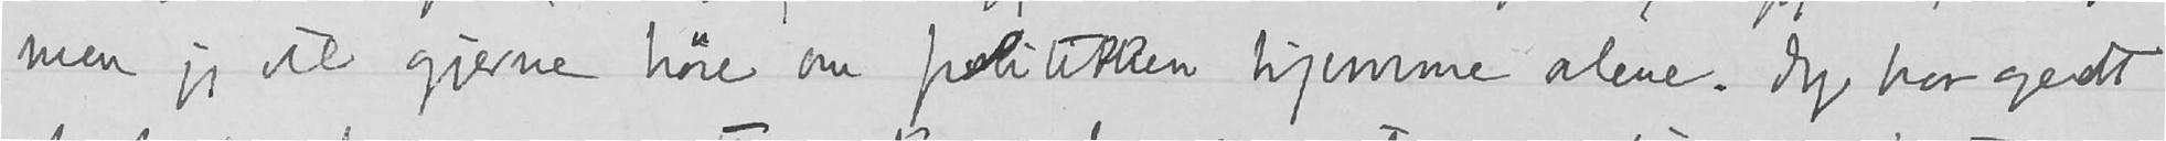men jeg vil gjerne høre om politikken hjemme alene. Jeg har godt
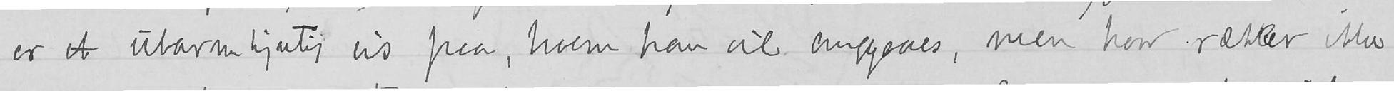er et ubarmhjertig vis paa, hvem han vil omgaaes, men han rækker ikke
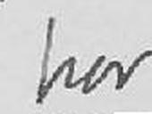har
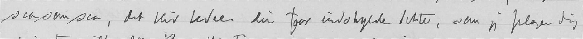saasomsaa, det blir bedre. Du faar undskylde dette, som jeg plager dig
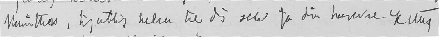Munthes, hjertelig hilsen til dig selv fra din hengivne Kitty
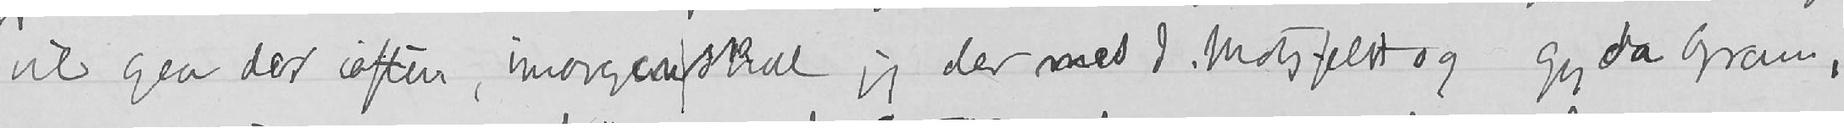vil gaa der iaften, imorgen skal jeg der med I. Motzfeldt og Gyda Gram,
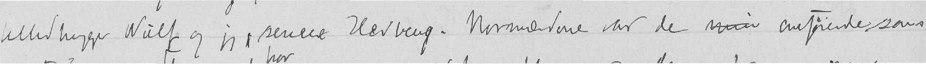billedhugger Wulf og jeg, senere Hedberg. Normændene var de min anførende som
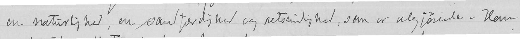en naturlighed, en sandfærdighed og retsindighed, som er velgjørende. Han
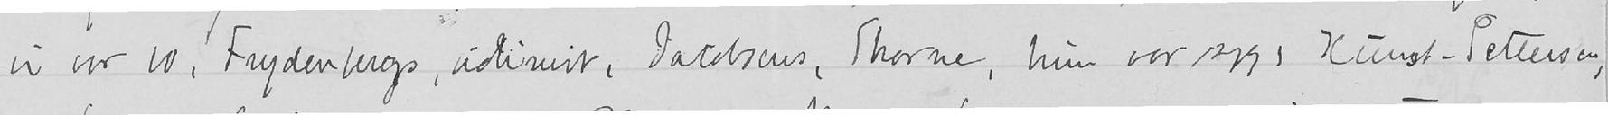vi var 10, Frydenbergs,  , Jacobsens, Thorne, hun var syg, Kunst-Pettersen,
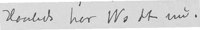Hvorledes har Ws det nu.
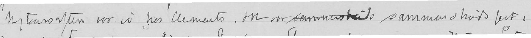Nytaarsaften var vi hos Clements. Det var sammenskuls sammenskudsfest,
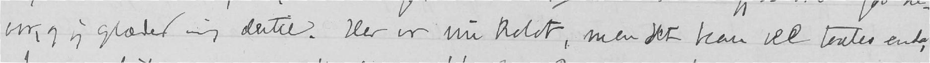vor, og jeg glæder mig dertil. Her er nu koldt, men det kan vel taales enda,
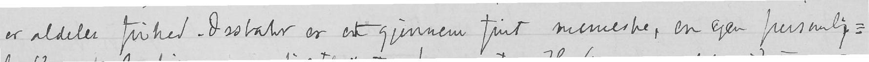er aldeles frihed. Ossbahr er et gjennem fint menneske, en egen personlig-
